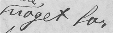noget for
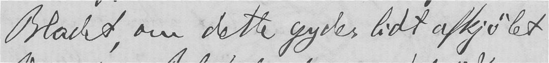Bladet om dette gyder lidt afkjølet
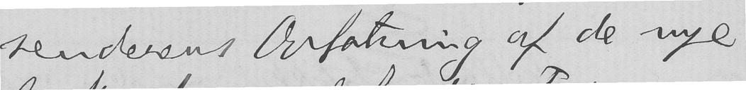senderens Opfatning af de nye
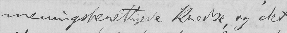meningsberettigede Kredse, og det
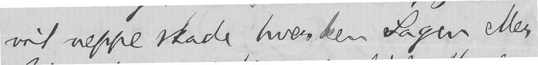vil neppe skade hverken Sagen eller
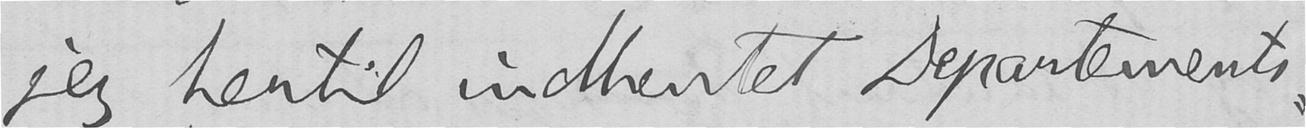jeg hertil indhentet Departements-
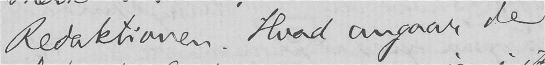Redaktionen. Hvad angaar de
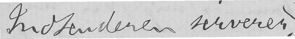Indsenderen serverer,
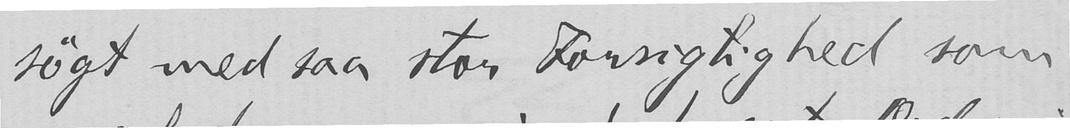søgt med saa stor Forsigtighed som
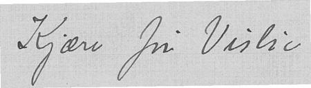Kjære fru Vislie
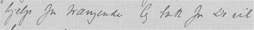hjelp for trængende. Og tak for De vil
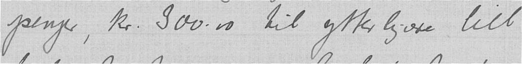penger, kr. 300.00 til ytterligere litt
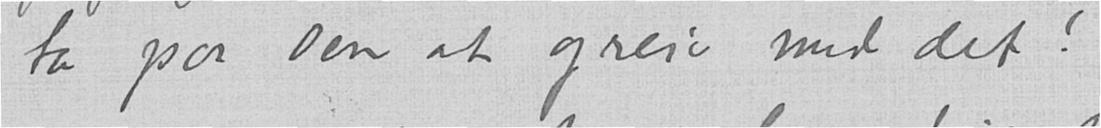ta paa Dem at greie med det!
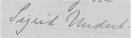Sigrid Undset.
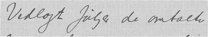Vedlagt følger de omtalte
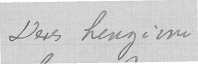Deres hengivne
